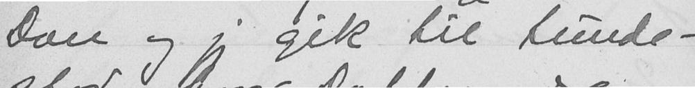Don og jeg gik til Lunde-
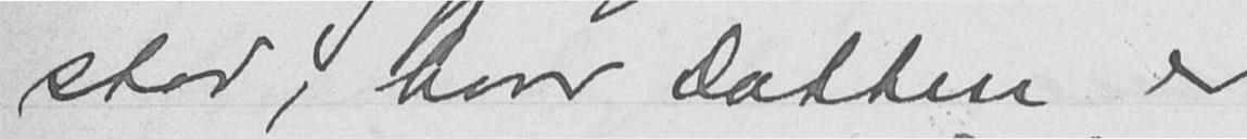stad, hvor Dotten er
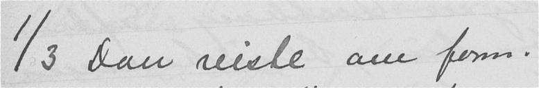1/3 Don reiste om form.
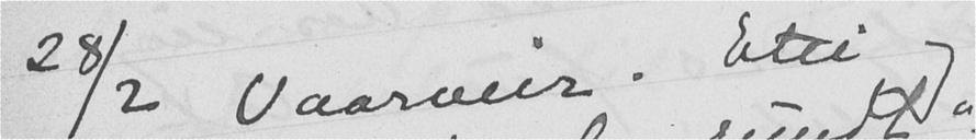28/2 Vaarveir. Etti og
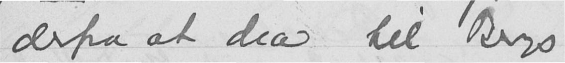derfra at dra til Bergs
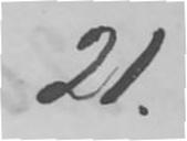21.
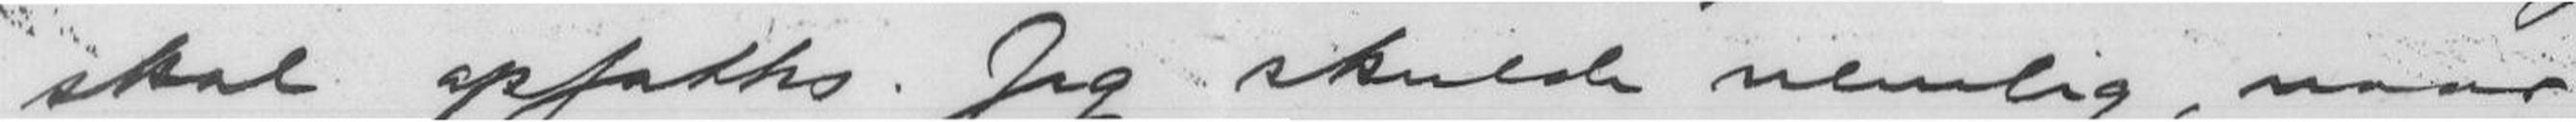skal opfattes. Jeg skulde nemlig, naar
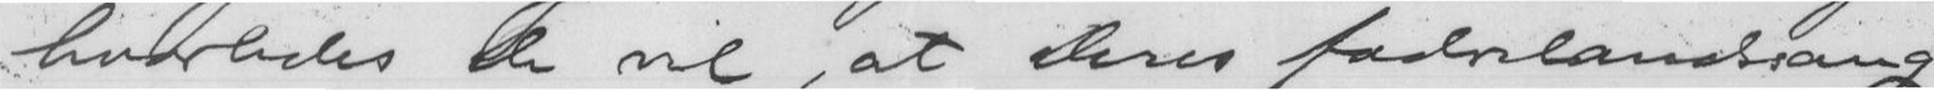hvorledes De vil, at Deres fædrelandssang
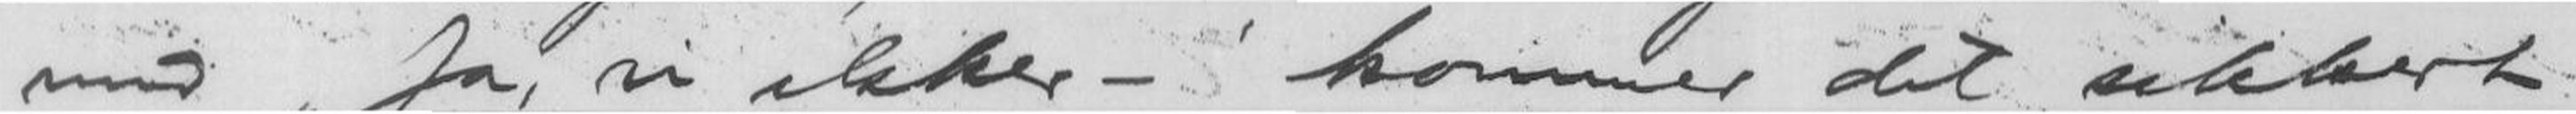med "Ja, vi elsker - kommer det sikkert
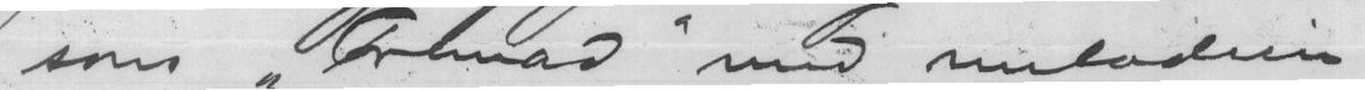sons Fremad med melodien
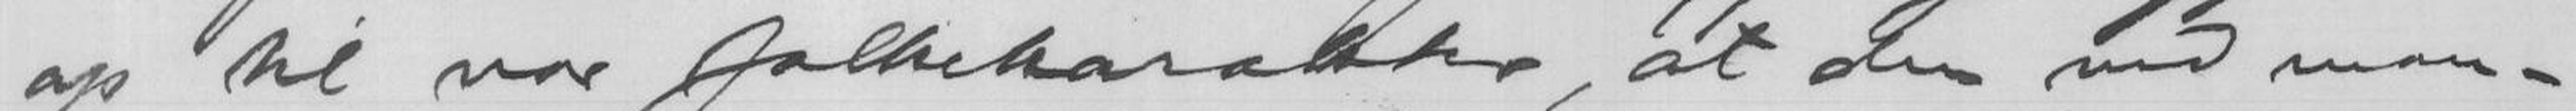op til vor folkekarakter, at den med man-
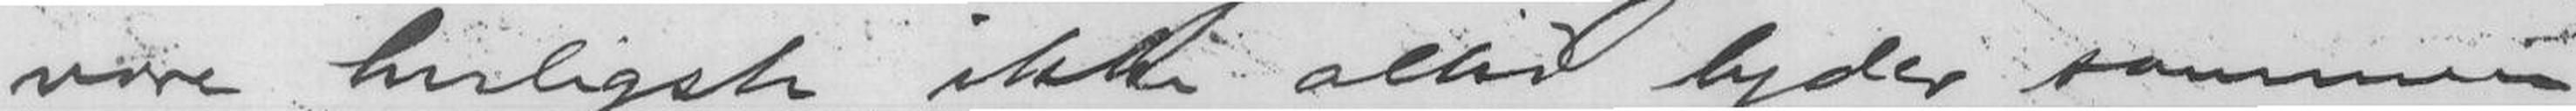vore herligste, ikke altid lyder sammen
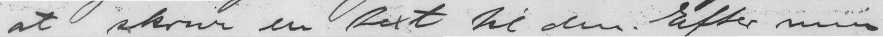at skrive en text til den. Efter min
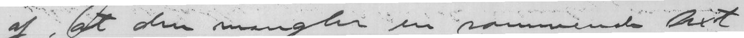af, at den mangler en samlende text.
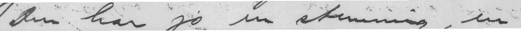Den har jo en stemning, en udslighed??,
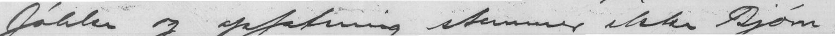følelse og opfatning stemmer ikke Bjørn-
